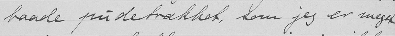- baade pudetrækket, som jeg er meget
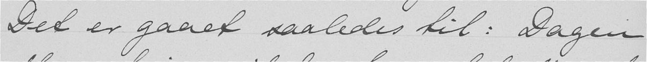Det er gaaet saaledes til: Dagen
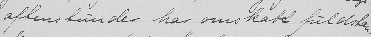aftenstunder har omskabt fuldstæn-
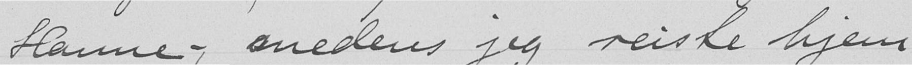Hanne -, medens jeg reiste hjem
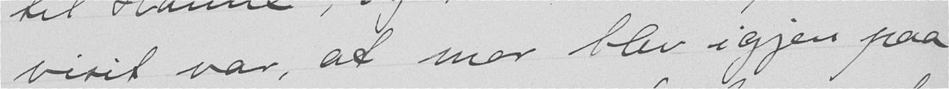visit var, at mor blev igjen paa
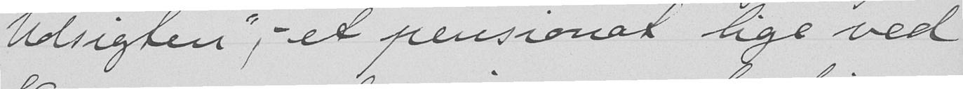"Udsigten", - et pensionat lige ved
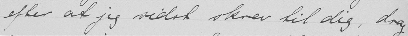efter at jeg sidst skrev til dig, drog
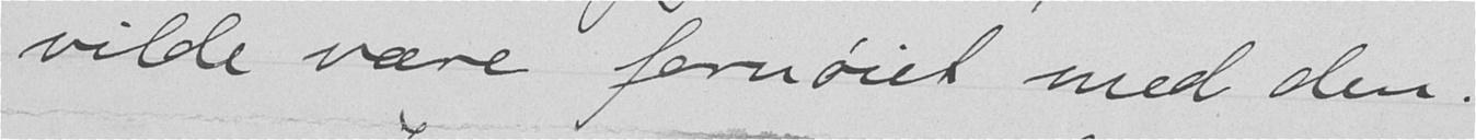vilde være fornøiet med den.
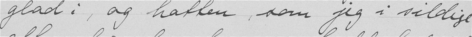glad i, og hatten, som jeg i sildige
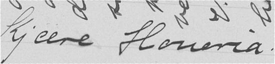Kjære Honoria!
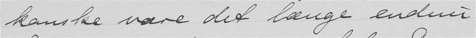kanske være det længe endnu.
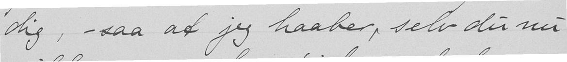dig, - saa at jeg haaber, selv du nu
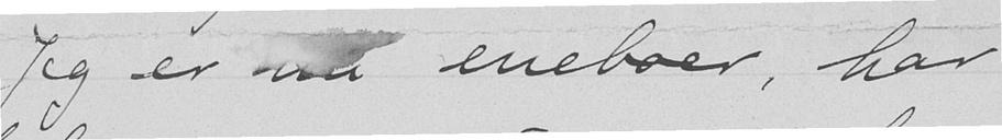Jeg er nu eneboer, har
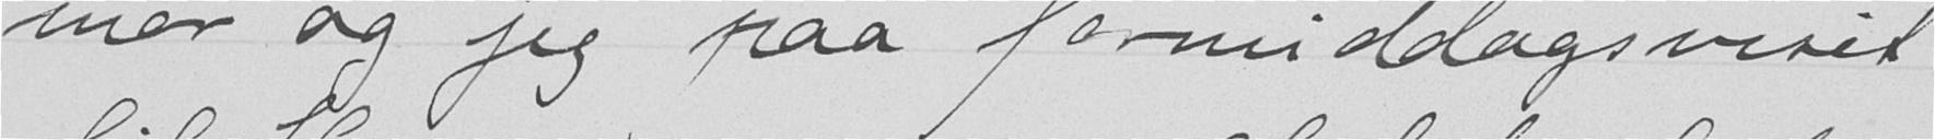mor og jeg paa formiddagsvisit
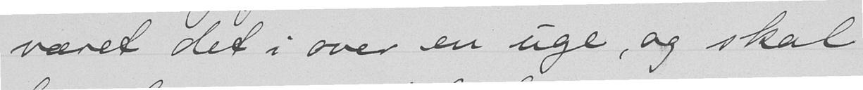været det i over en uge, og skal
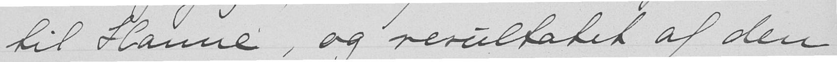til Hanne, og resultatet af den
